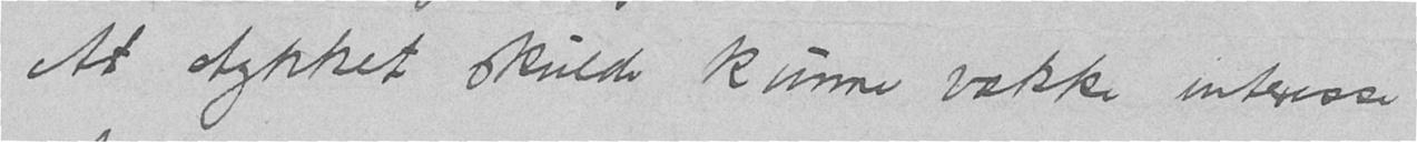At stykket skulde kunne vække interesse
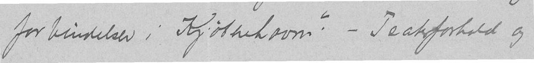forbindelser i Kjøbenhavn? – Teaterforhold og
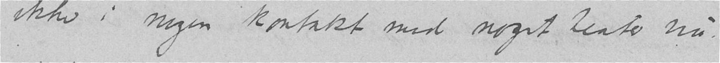ikke i nogen kontakt med noget teater nu.
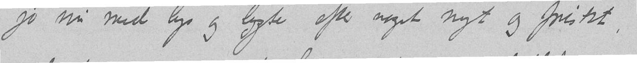jo nu med lys og lygte efter noget nyt og friskt
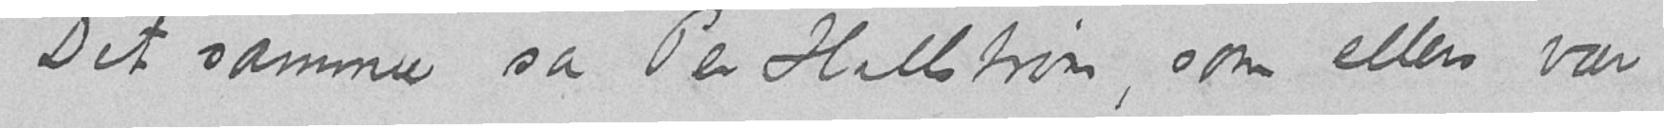Det sammee sa Per Hellstrøm, som ellers var
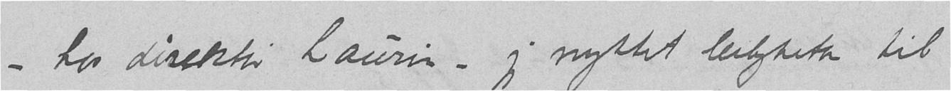- hos direktør Laurin - jeg nyttet leiligheten til
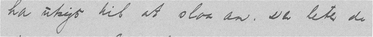ha utsigt til at slaa an. Der leter de
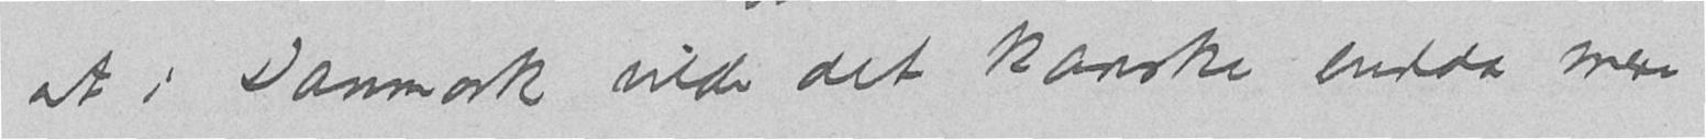at i Danmark vilde det kanske endda mere
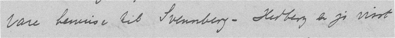bare henvise til Svennberg – Hedberg er jo visst
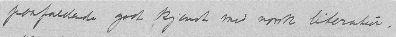paafaldende godt kjendt med norsk literatur.
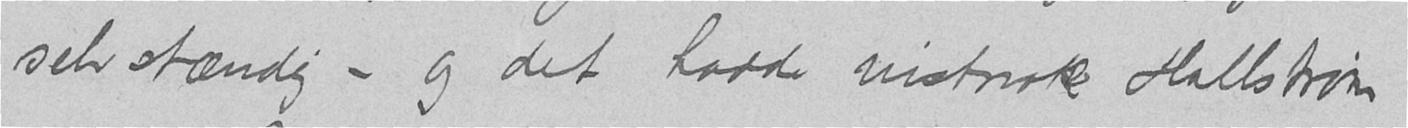selvstændig – og det hadde vistnok Hallstrøm
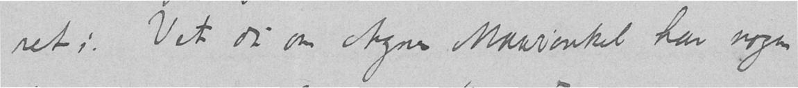ret i. Vet du om Agnes Mowinkel har nogen
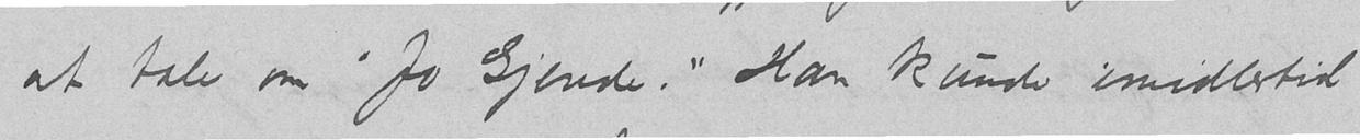at tale om "Jo Gjende." Han kunde imidlertid
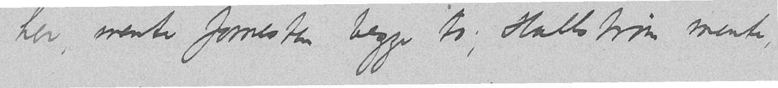her mente forresten begge to; Hallstrøm mente,
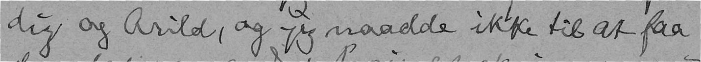dig og Arild, og jeg naadde ikke til at faa
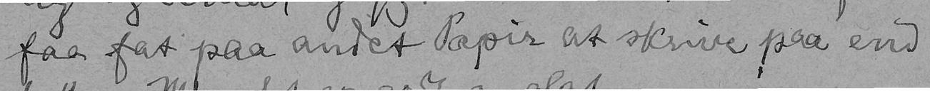faa fat paa andet Papir at skrive paa end
